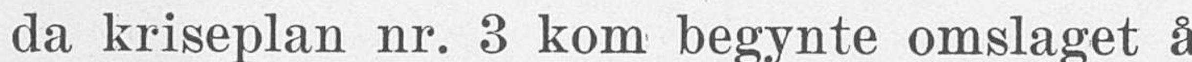da kriseplan nr. 3 kom begynte omslaget å
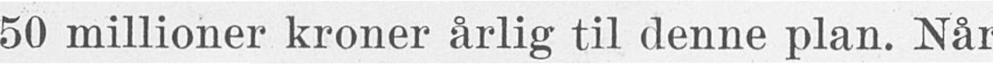50 millioner kroner årlig til denne plan. Når
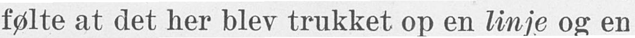følte at det her blev trukket op en linje og en
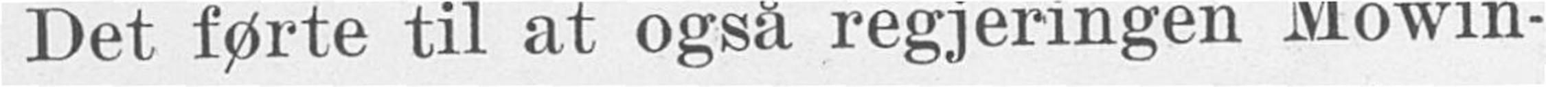Det førte til at også regjeringen Mowin-
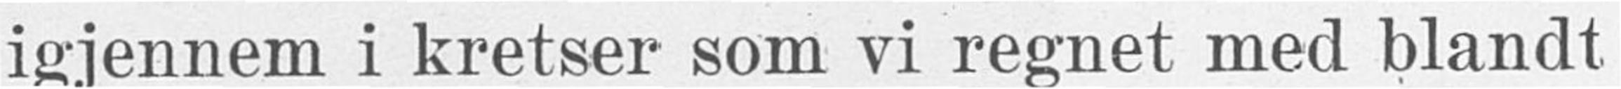igjennem i kretser som vi regnet med blandt
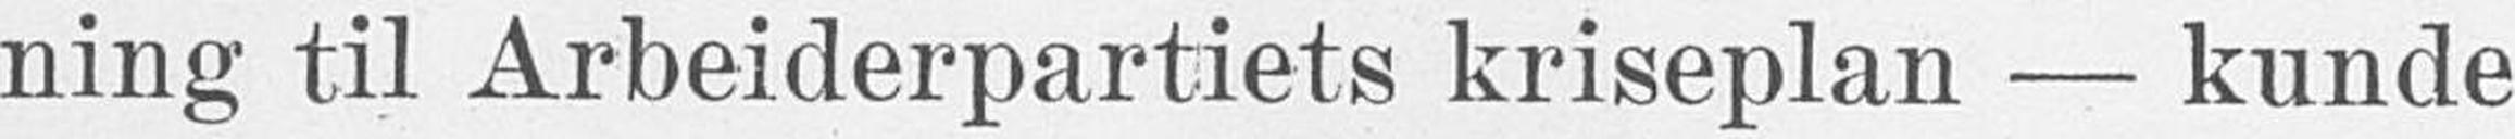ning til Arbeiderpartiets kriseplan - kunde
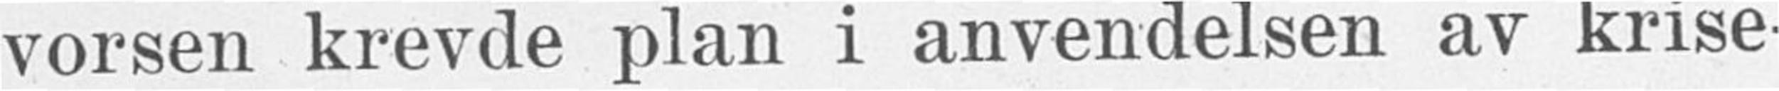vorsen krevde plan i anvendelsen av krise-
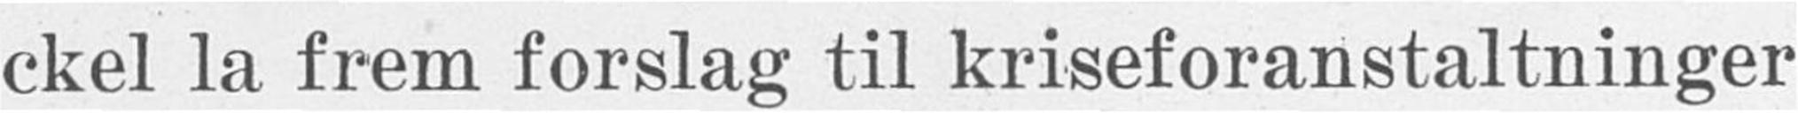ckel la frem forslag til kriseforanstaltninger
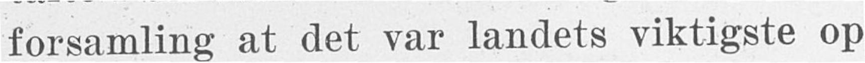forsamling at det var landets viktigste op-
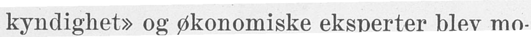kyndighet" og økonomiske eksperter blev mo-
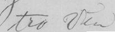tro Ven
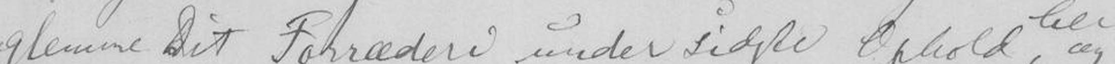glemme Dit Forræderi under sidste Ophold, og
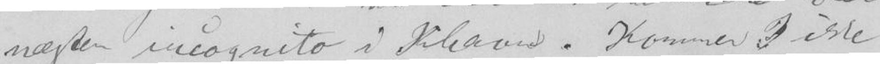næsten incognito i Khavn. Kommer I ikke
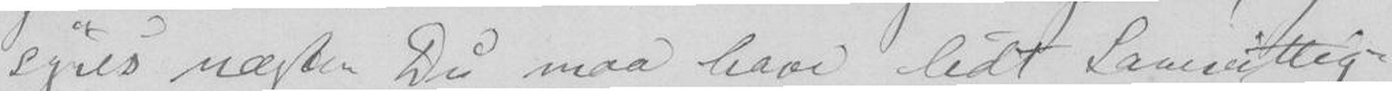synes næsten Du maa have lidt Samvittig-
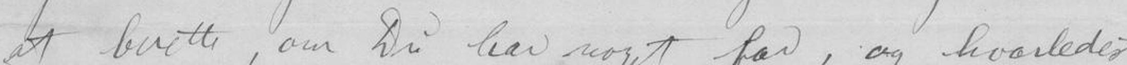at berette, om Du har noget fad, og hvorledes
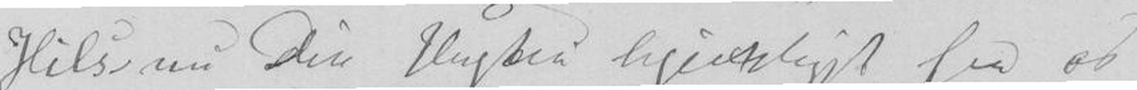Hils nu Din Hustru hjerteligst fra os
